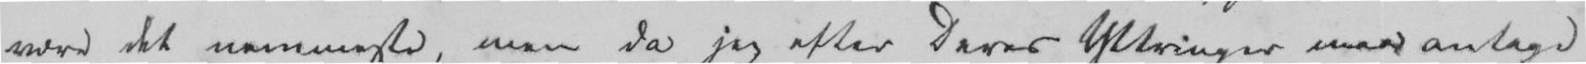være det nemmeste, men da jeg efter Deres Yttringer maa antage
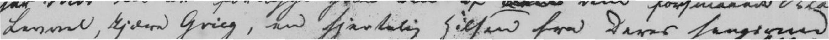Levvel, kjære Grieg, en hjertelig Hilsen fra Deres hengivne
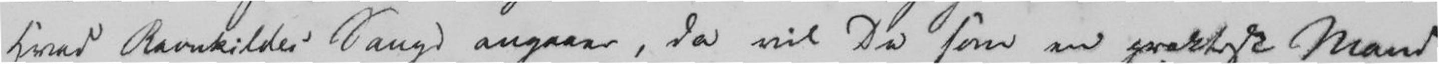Hvad Ravnkildes' Sange angaar, da vil De som en præktig Mand
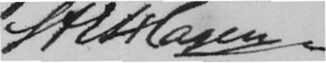S A E Hagen
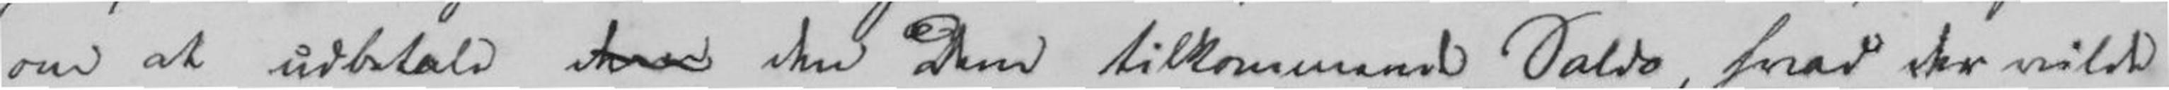om at udbetale dem den Dem tilkommende Saldo, hvad der vilde
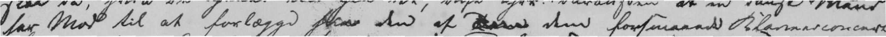har Mod til at forlægge han den af Dem dem forsmaaede Klaveerconcert.
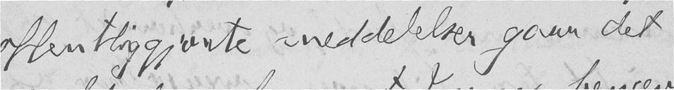offentliggjorte meddelelser gaar det
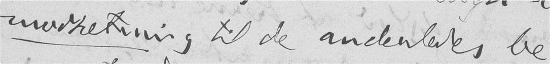modsætning til de anderledes be-
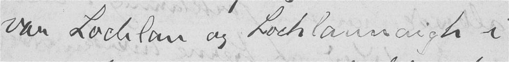var Lochlan og Lochlannaigh i
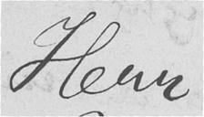Herr
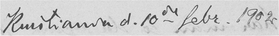Kristiania d. 10de febr. 1902.
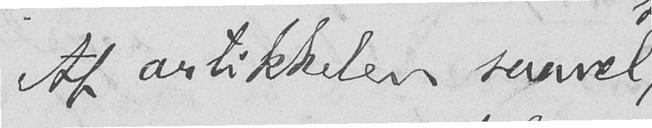Af artikkelen saavel
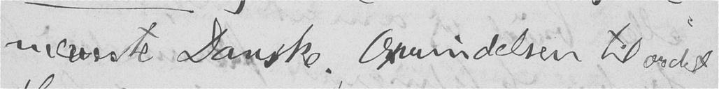nævnte Danske. Oprindelsen til ordet
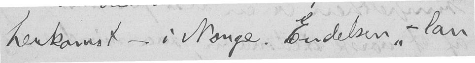herkomst - i Norge. Endelsen "-lan"
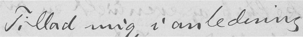Tillad mig, i anledning
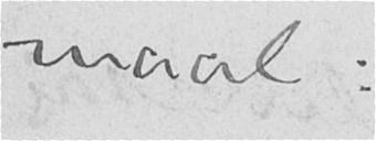maal:
